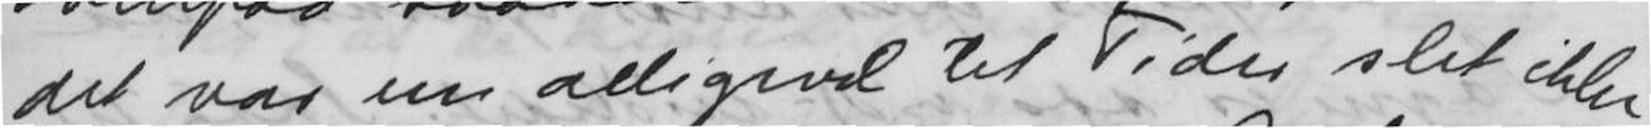det var nu alligevel til Tider slet ikke
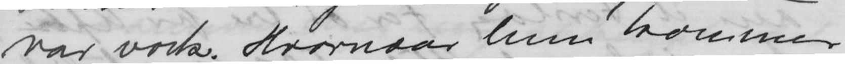var vaek. Hvornaar hun kommer
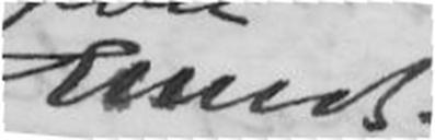Ellen B.
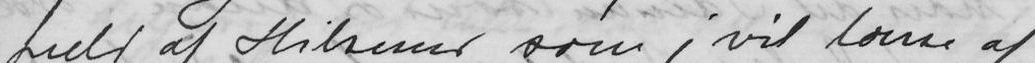fuld af Hilsener som j vil laesse af
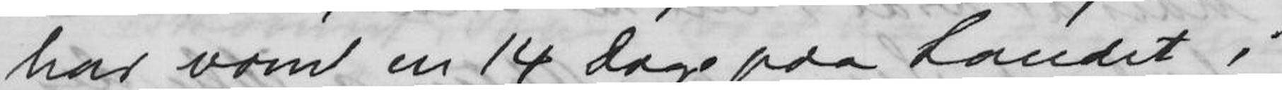har vaeret en 14 Dage paa Landet i
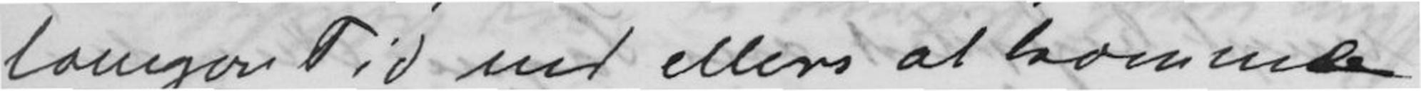laenger Tid end ellers at komme
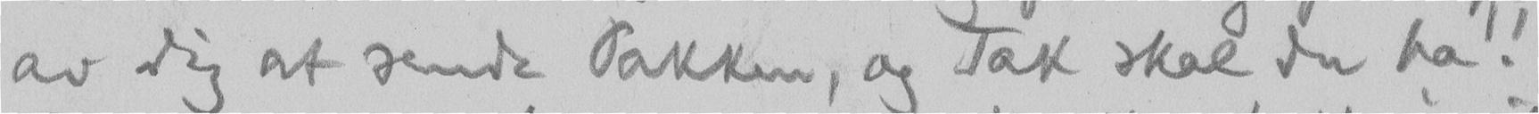av dig at sende Pakken, og Tak skal du ha!
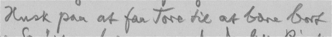Husk paa at faa Tore til at bære bort
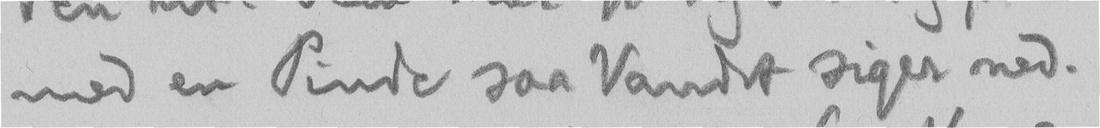med en Pinde saa Vandet siger ned.
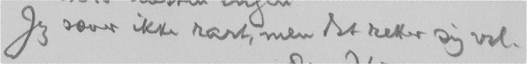Jeg sover ikke rart, men det retter sig vel.
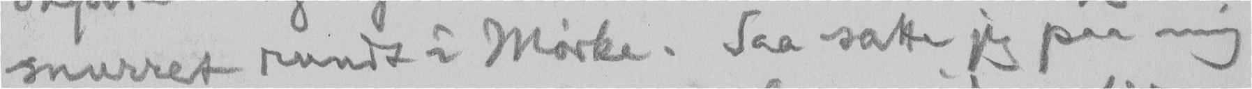snurret rundt i Mørke. Saa satte jeg paa mig
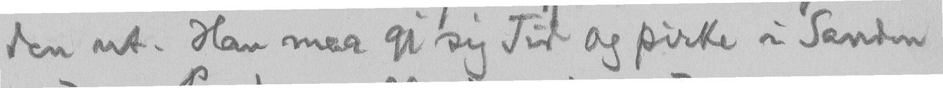den ud. Han maa gi sig Tid og pirke i Sanden
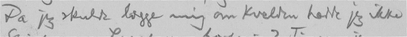Da jeg skulde lægge mig om Kvelden hadde jeg ikke
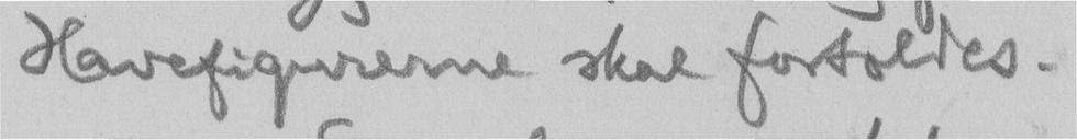Havefigurerne skal fortoldes.
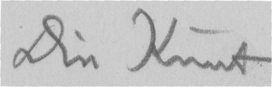Din Knut
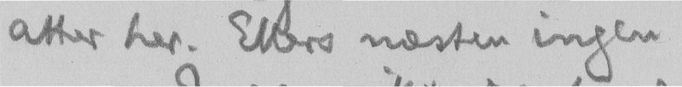atter her. Ellers næsten ingen
